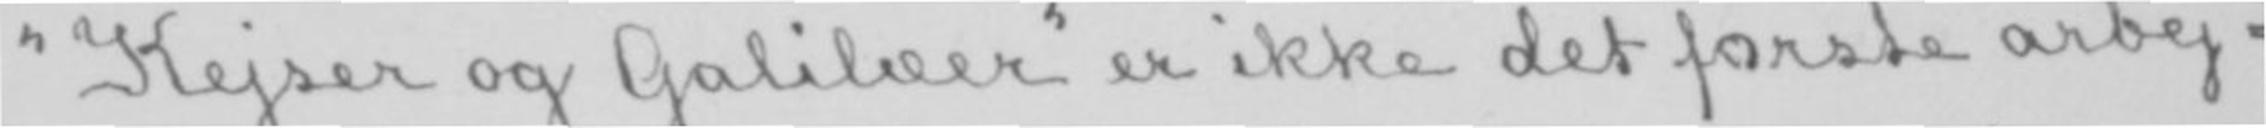«Kejser og Galilæer» er ikke det første arbej-
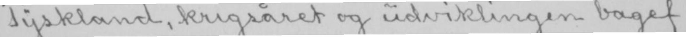Tyskland, krigsåret og udviklingen bagef-
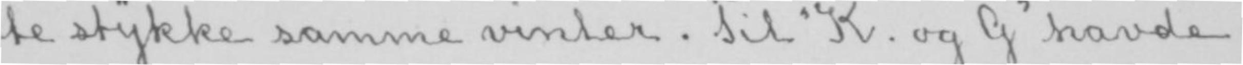te stykke samme vinter. Til «K. og G» havde
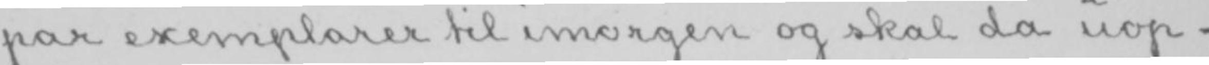par exemplarer til imorgen og skal da uop-
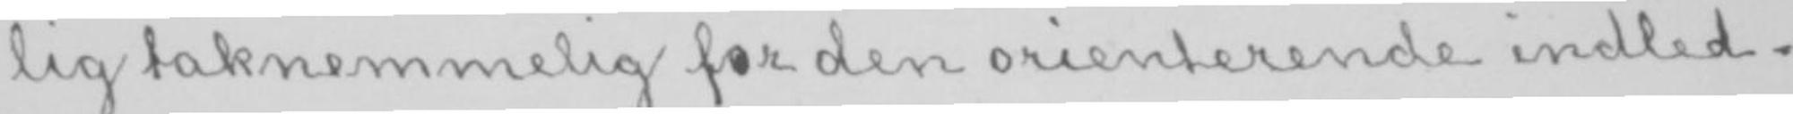lig taknemmelig for den orienterende indled-
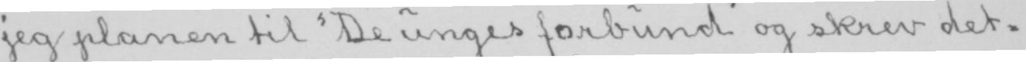jeg planen til «De unges forbund» og skrev det-
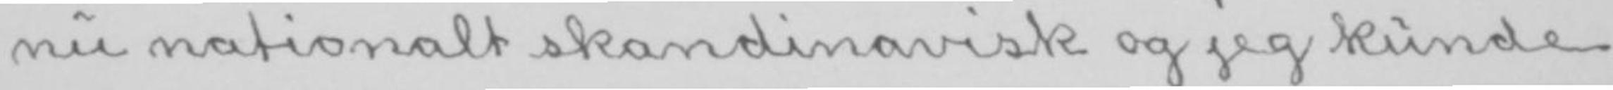nu nationalt skandinavisk og jeg kunde
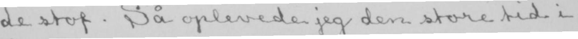de stof. Så oplevede jeg den store tid i
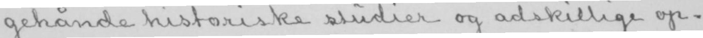gehånde historiske studier og adskillige op-
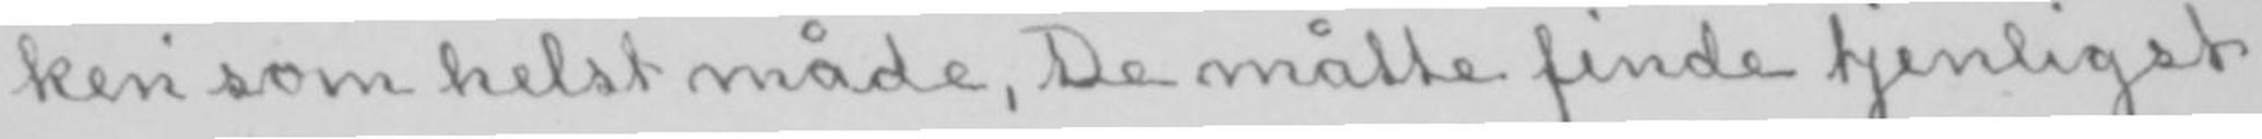ken som helst måde, De måtte finde tjenligst
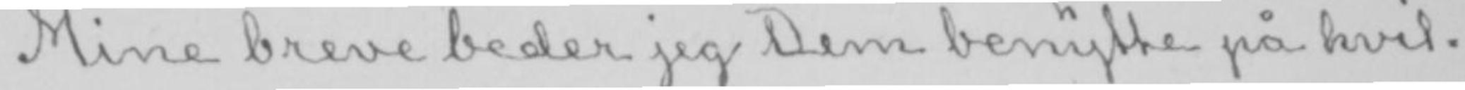Mine breve beder jeg Dem benytte på hvil-
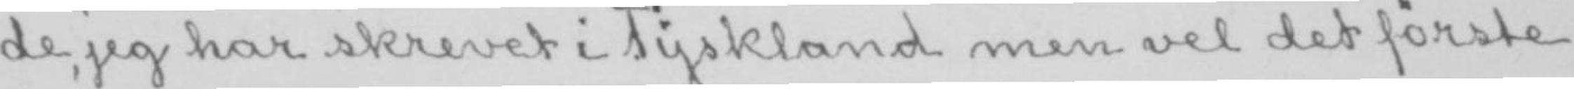de, jeg har skrevet i Tyskland men vel det første
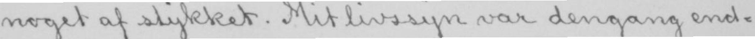noget af stykket. Mit livssyn var dengang end-
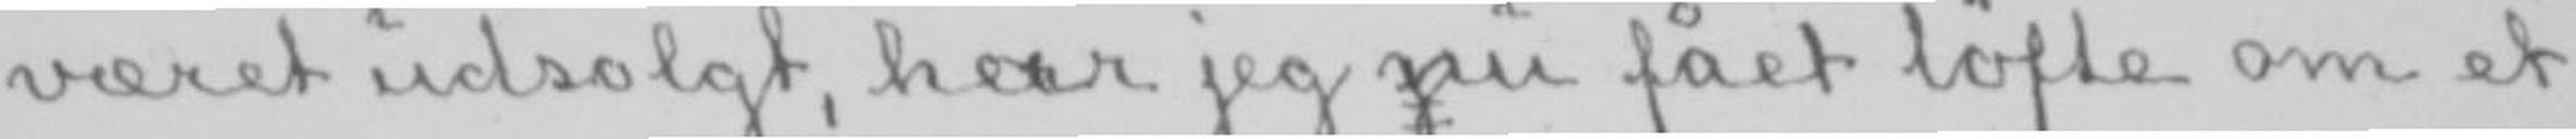været udsolgt, herar jeg jnu fået løfte om et
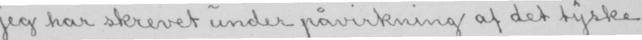jeg har skrevet under påvirkning af det tyske
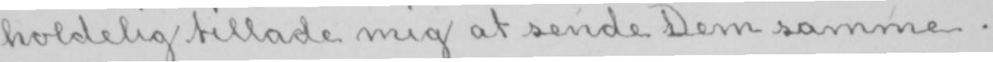holdelig tillade mig at sende Dem samme.
